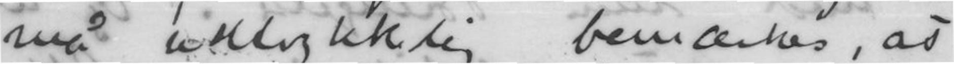må utrykkelig bemærkes, at
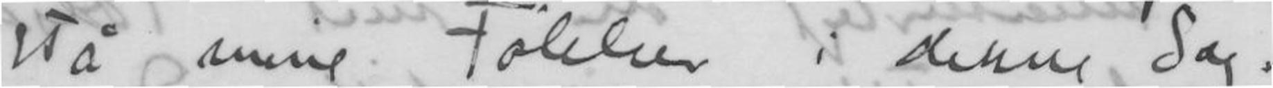stå mine Følelser i denne Sag.
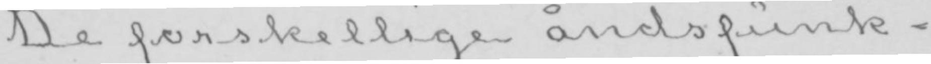De forskellige åndsfunk-
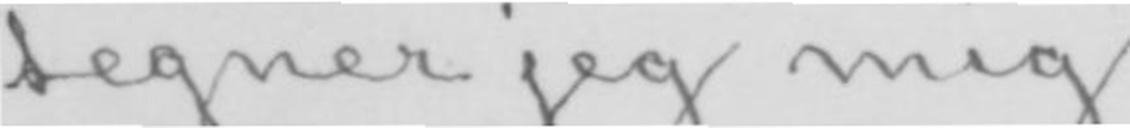tegner jeg mig
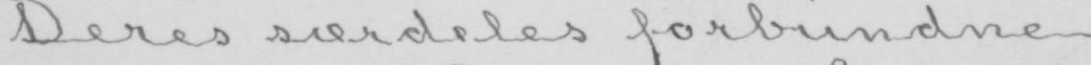Deres særdeles forbundne
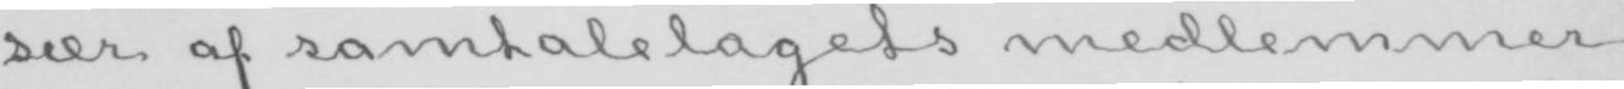sær af samtalelagets medlemmer
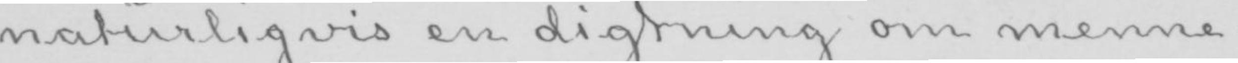naturligvis en digtning om menne-
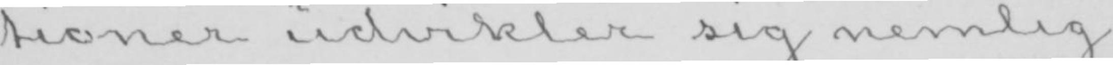tioner udvikler sig nemlig
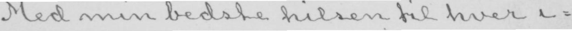Med min beste hilsen til hver i-
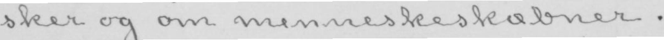sker og om menneskeskæbner.
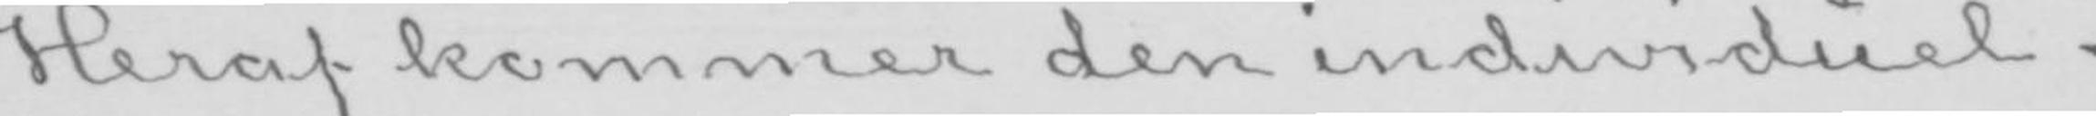Heraf kommer den individuel-
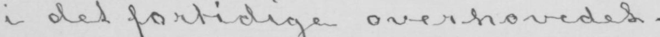i det fortidige overhovedet.
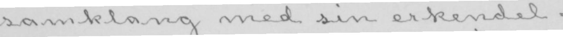samklang med sin erkendel-
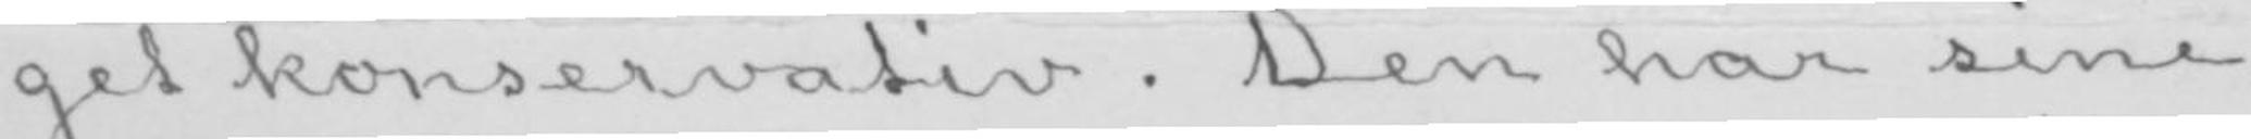get konservativ. Den har sine
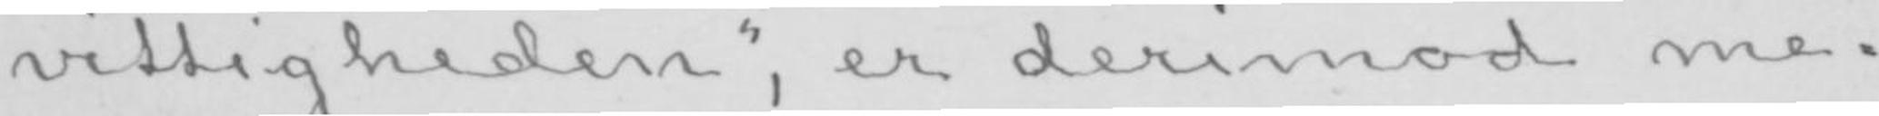vittigheden», er derimod me-
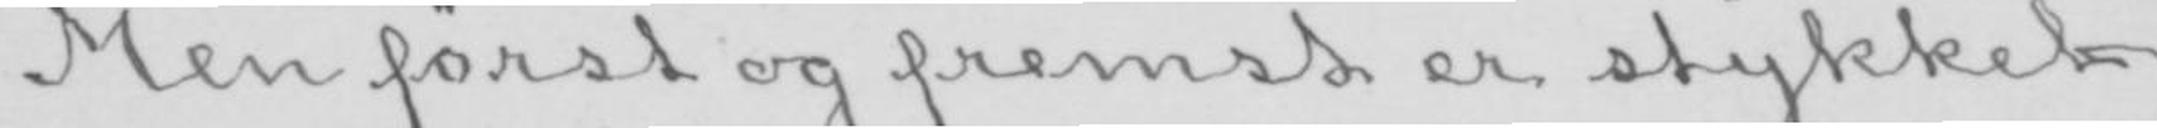Men først og fremst er stykket
